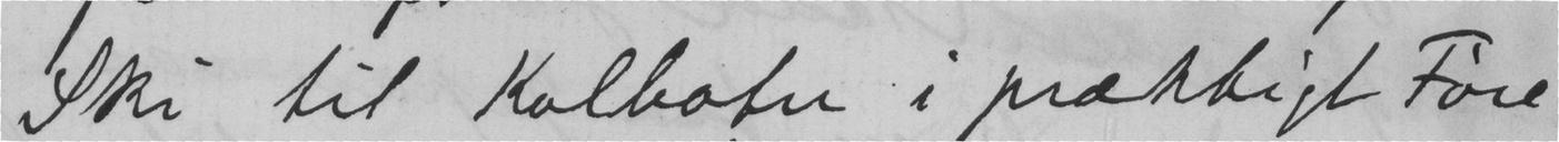Ski til Kolbotn i præktigt Føre
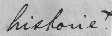historie.
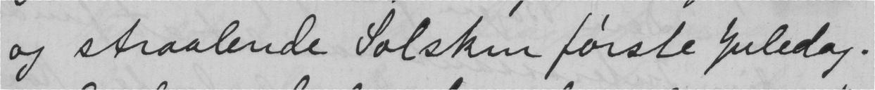og straalende Solskin første Juledag.
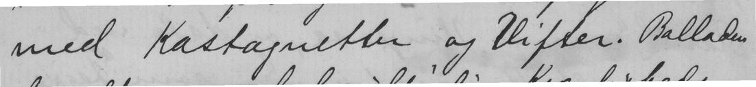med Kastagnetter og Vifter. Balladen
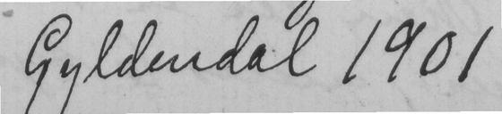Gyldendal 1901
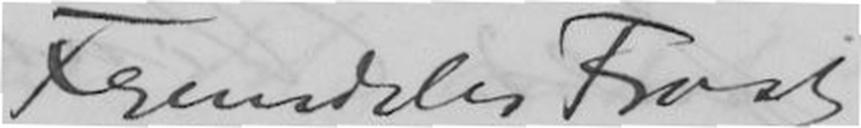Fremdeles Frost
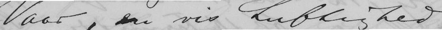Vaar, en vis Luftighed
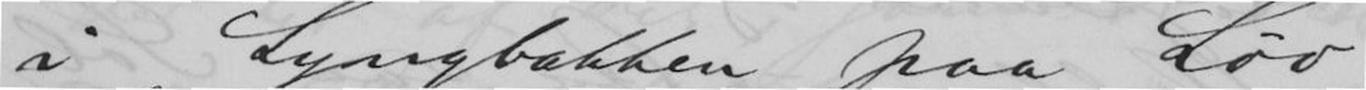i Lyngbakken paa Løv-
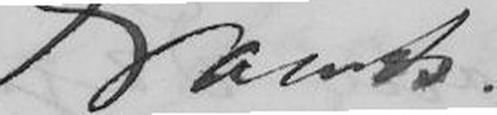Frants.
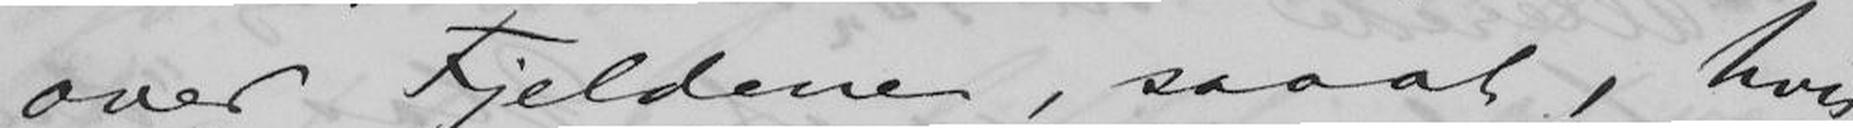over Fjeldene, saaat, hvis
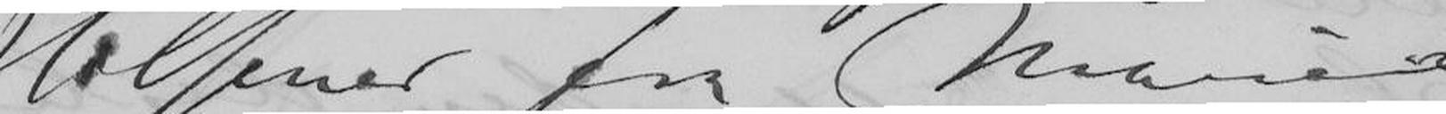Hilsener fra Marie
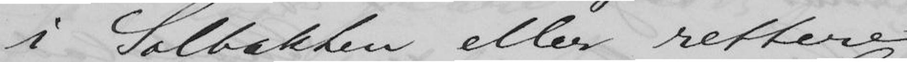i Solbakken eller rettere
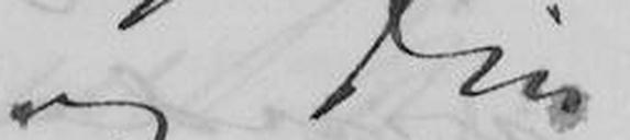og Din
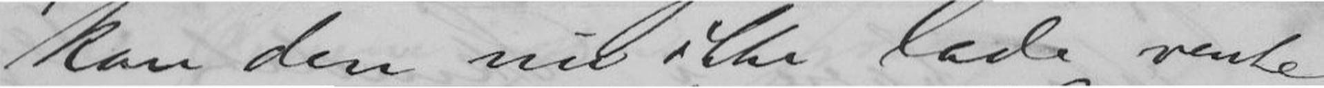kan den nu ikke lade vente
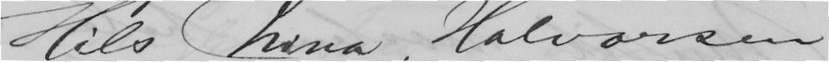Hils Nina, Halvorsen
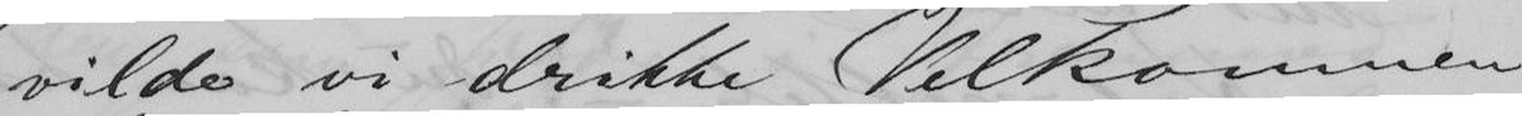vilde vi drikke Velkommen
